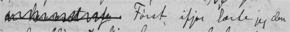Først ifjor lærte jeg den
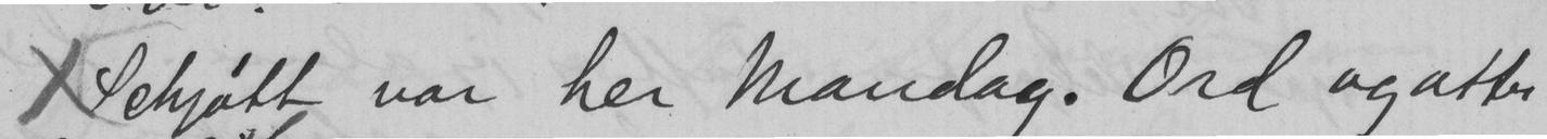x Schjøtt var her mandag. Ord og atter
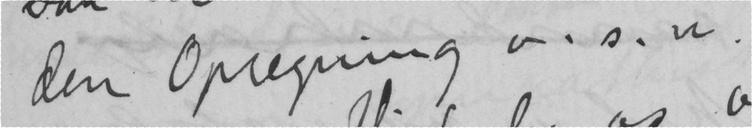den Opregning o. s. v.
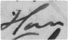Han
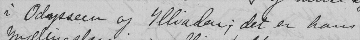i Odysseen og Illiaden; det er hans
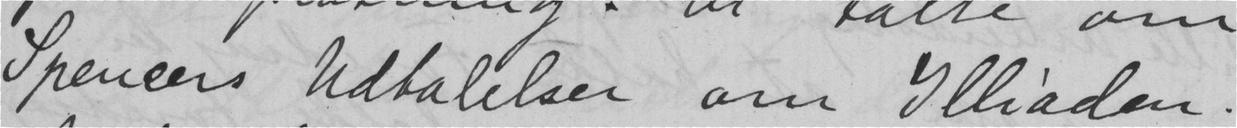Spencers Udtalelser om Illiaden.
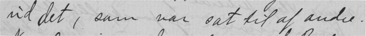ud det, som var sat til af andre.
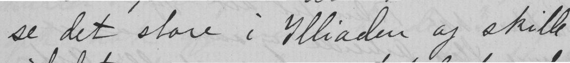se det store i Illiaden og skille
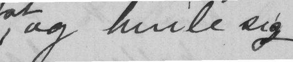og hvile sig
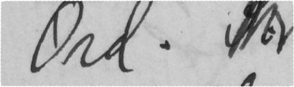Ord.
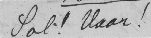Sol! Vaar!
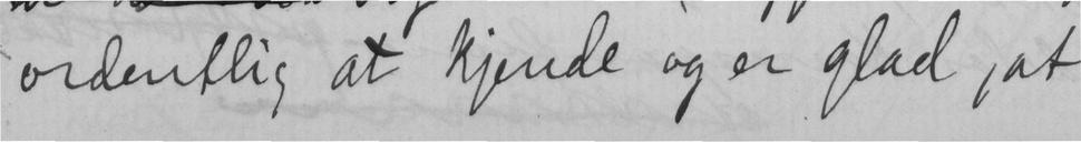ordentlig at kjende og er glad, at
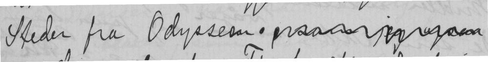Steder fra Odysseen.
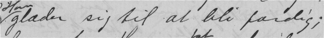glæder sig til at bli færdig;
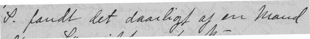S. fandt det daarligt af en Mand
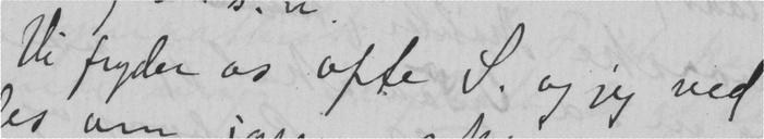Vi fryder os ofte S. og jeg ved
